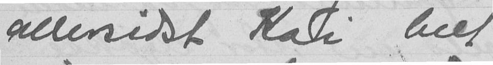allersidst Kai helt
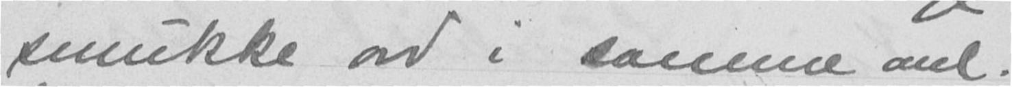smukke ord i samme anl.
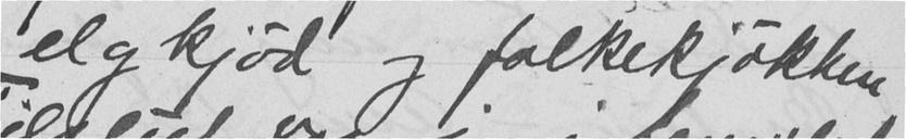elgkjød og folkekjøkken.
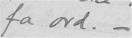Ja ord. -
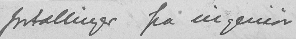fortællinger fra ingeniør
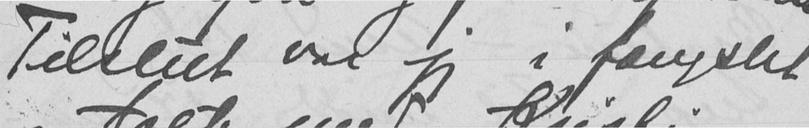Tilslut var jeg i fængslet
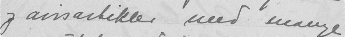og avisartikler med mange
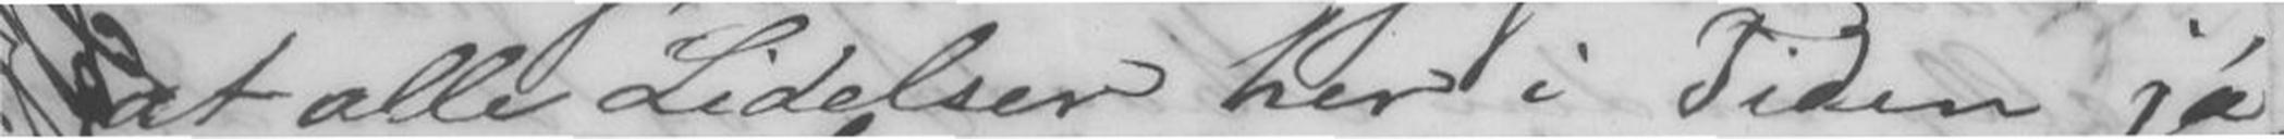at alle Lidelser her i Tiden, ja
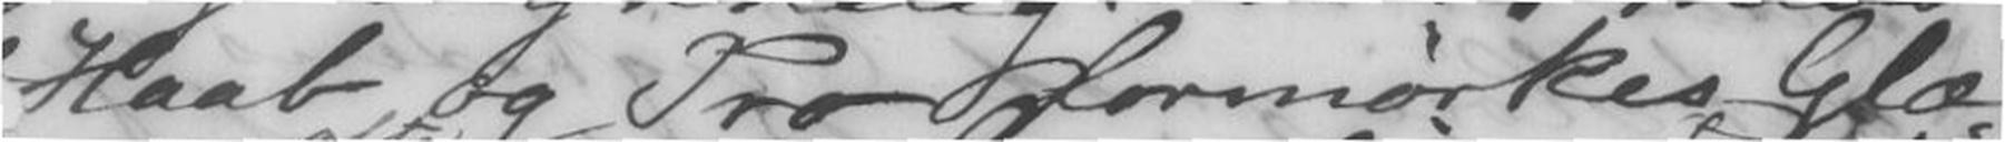Haab og Tro formørkes Glæ-
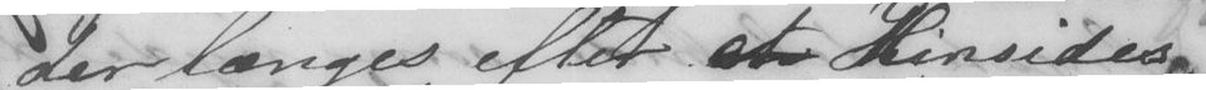der længes efter et Hinsides,
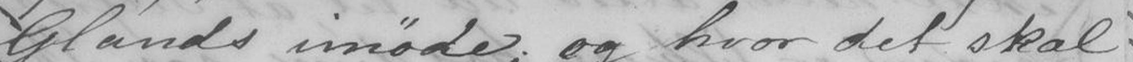Glands imøde og hvor det skal
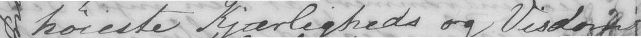høieste Kjærligheds og Visdoms
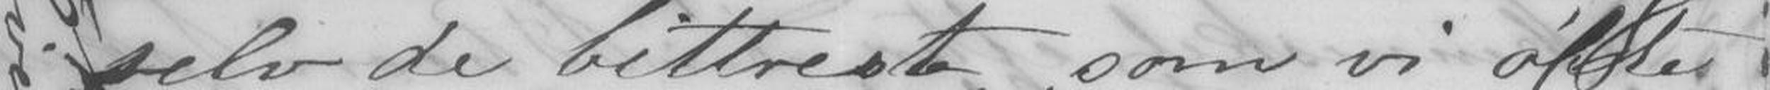selv de bittreste, som vi oftes
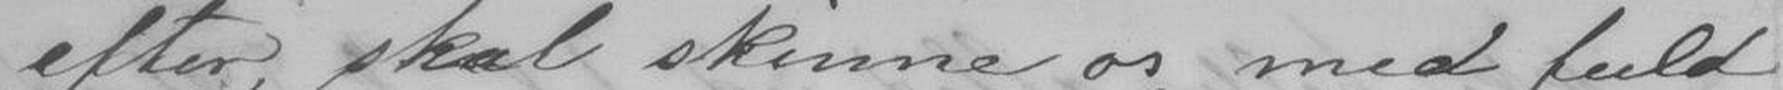efter, skal skinne os med fuld
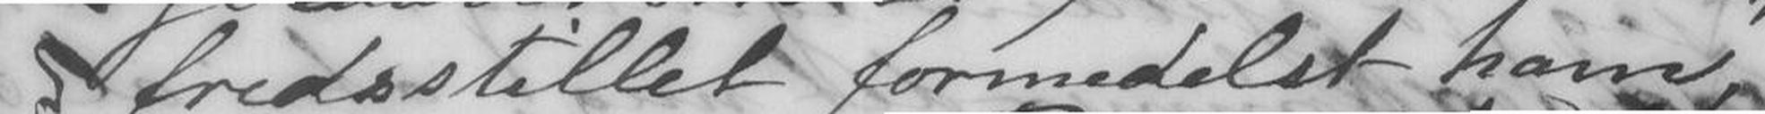fredsstillet formedelst ham
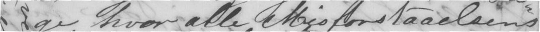ge, hvor alle Misforstaaelsens
